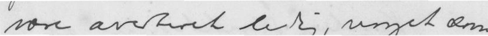være averteret ledig, noget som
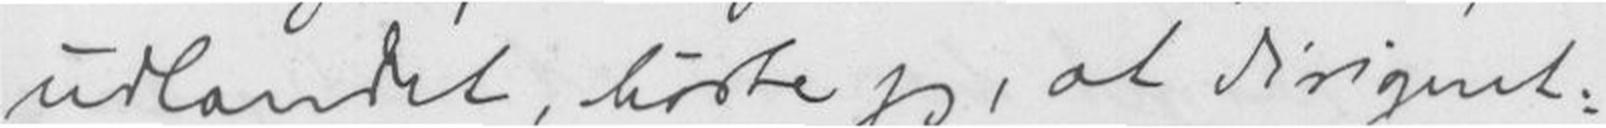udlandet, hørte jeg, at dirigent-
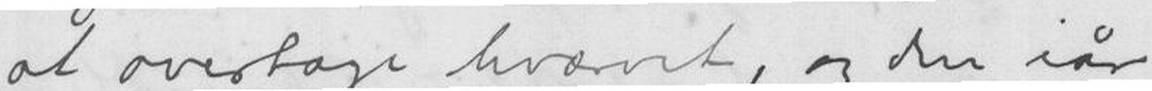at overtage hvorvit, og den iår
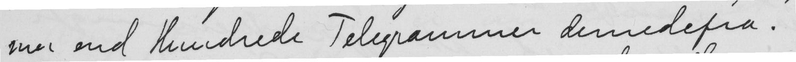mer end Hundrede Telegrammer dernedefra.
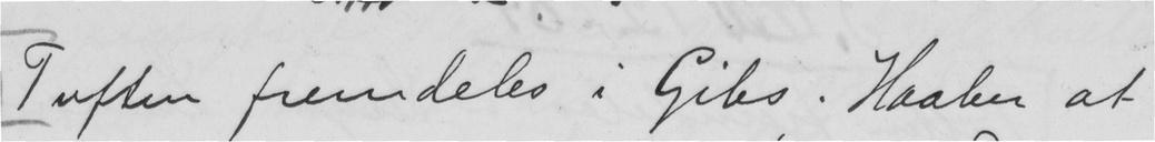Tuften fremdeles i Gibs. Haaber at
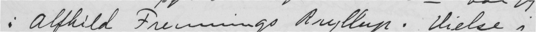i Alfhild Fremnings Bryllup. Vielse i
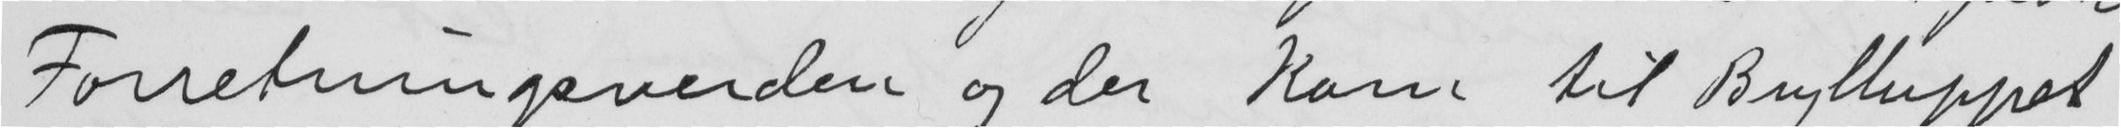Forretningsverden og der kom til Brylluppet
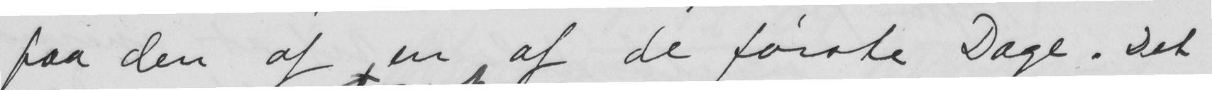faa den af en af de første Dage. Det
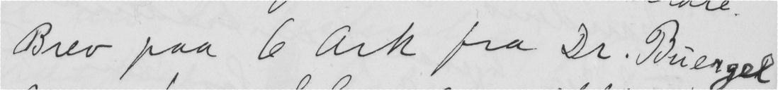Brev paa 6 Ark fra Dr. Buergel
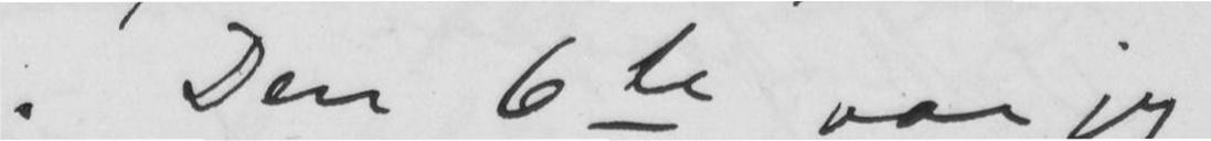. Den 6te var jeg
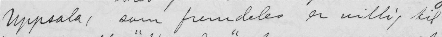Uppsala, som fremdeles er villig til
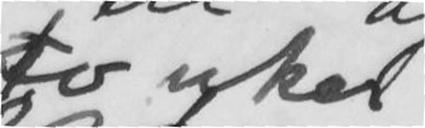to uker
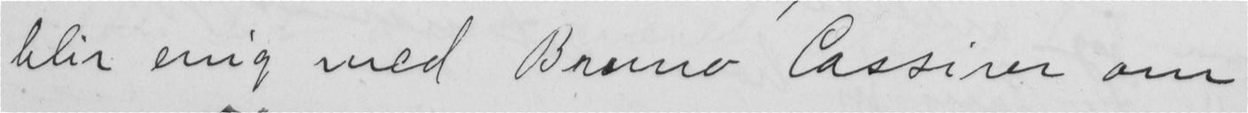blir enig med Bruno Cassirer om
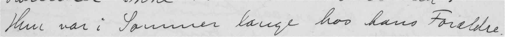Hun var i Sommer længe hos hans Forældre.
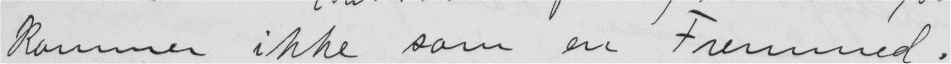kommer ikke som en Fremmed.
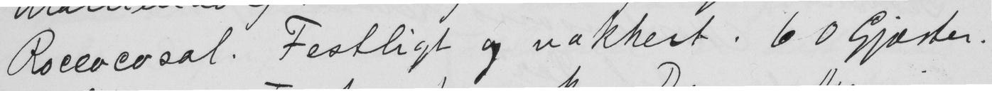Roccocosal. Festligt og vakkert. 60 Gjæster.
